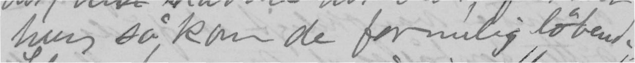hun så kom de formelig løbende.
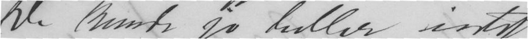De kunde jo heller intet
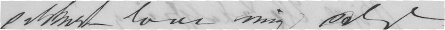sikkert love mig selv
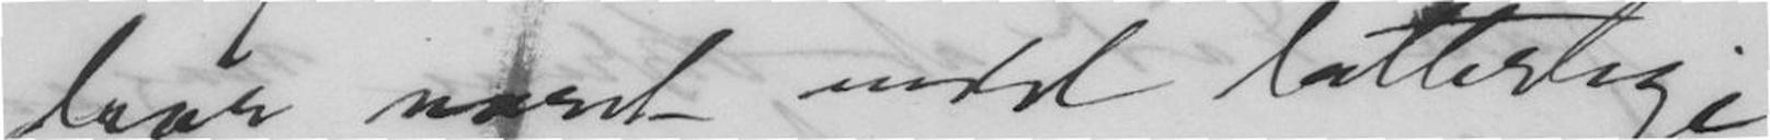har været endel latterlige
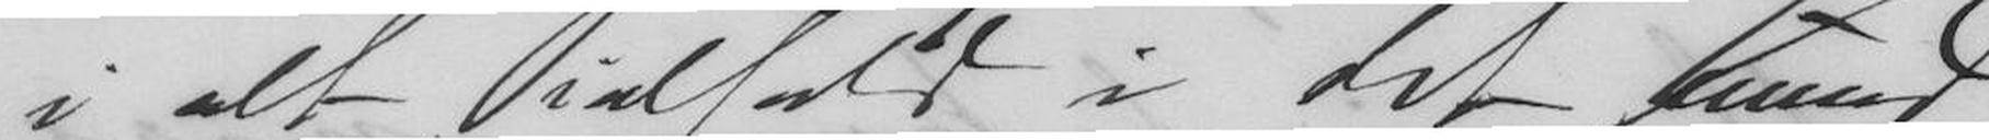i alt, ialfald i det uund-
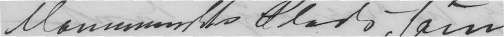Monumentets Plads, som
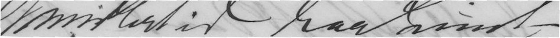Imidlertid har Smidt
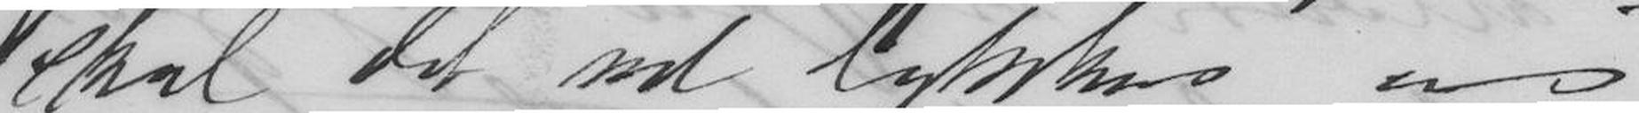skal det vel lykkes os
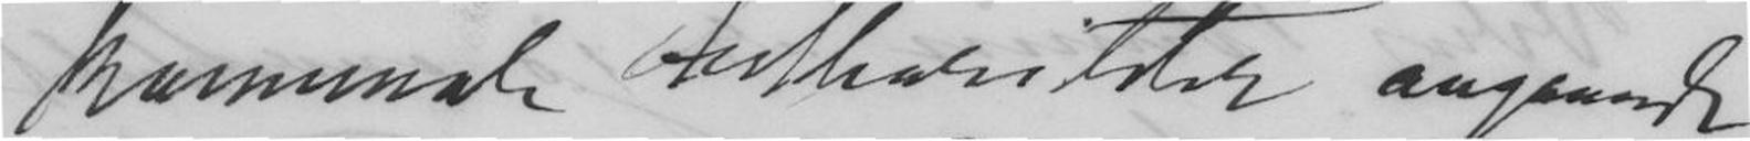komunale Festhariteter angaaende
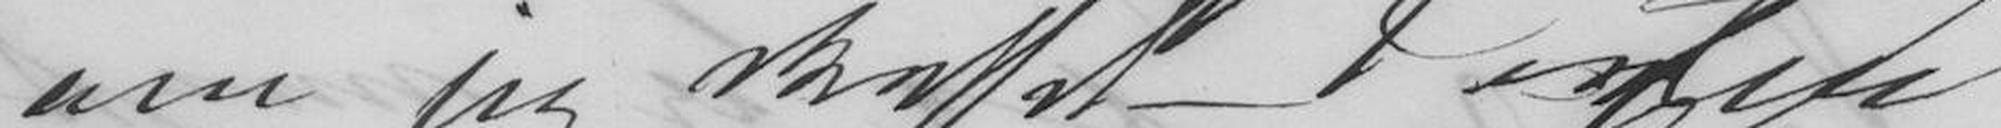om jeg skaffet Texten
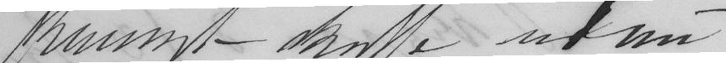kunnet skaffe endnu.
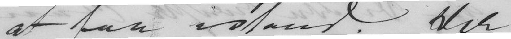at faa istand. Der
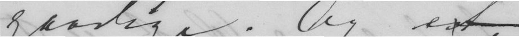gaaelige. Og et
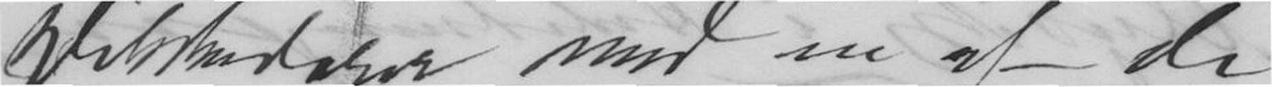Dikkedarer med en af de
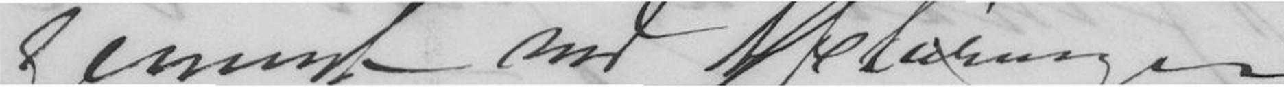gement ved Afsløringen
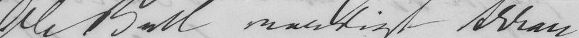Ole Bull værdigt Arran-
